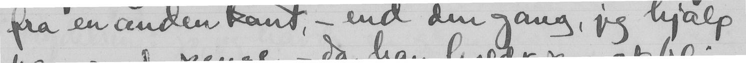fra en anden kant, - end den gang, jeg hjalp
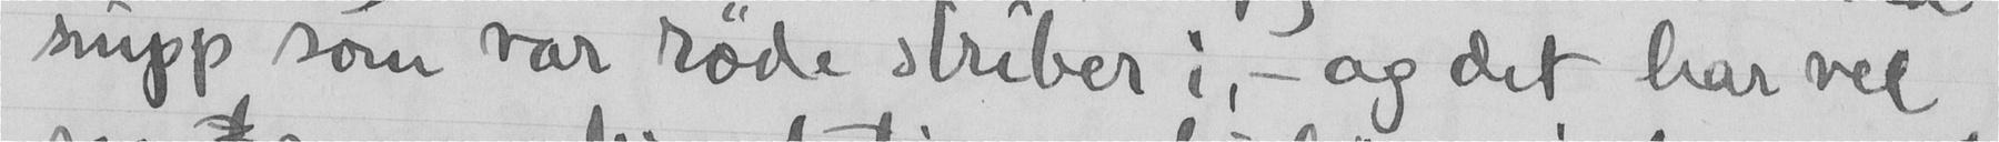snipp som var røde striber i, - og det har vel
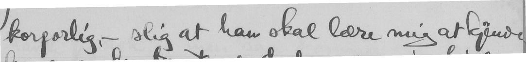korperlig, - slig at han skal lære mig at kjende
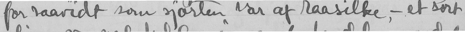for saavidt som sjorten var af raasilke, - et sort
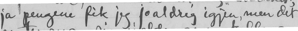ja pengene fik jeg jo aldrig igjen, men det
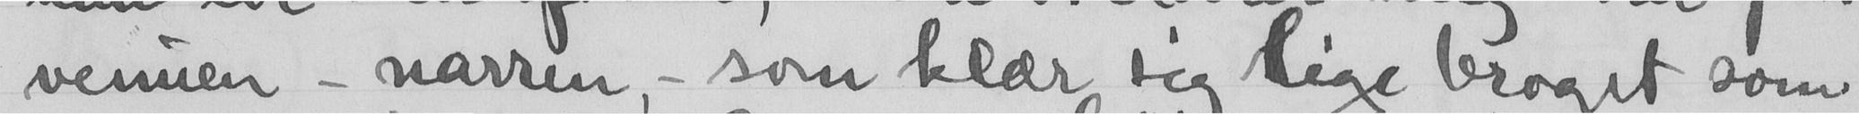venuen - narren, - som klær sig lige broget som
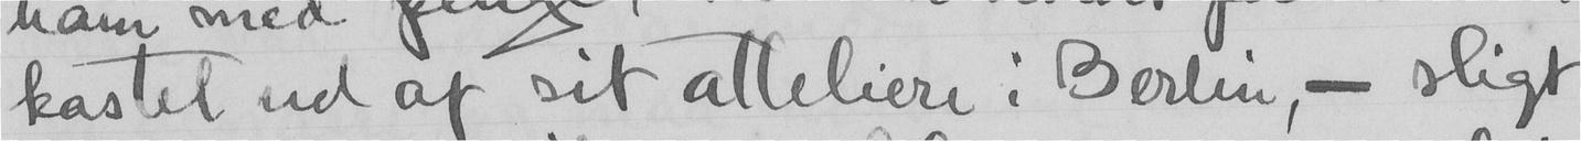kastet ud af sit atteliere i Berlin, - sligt
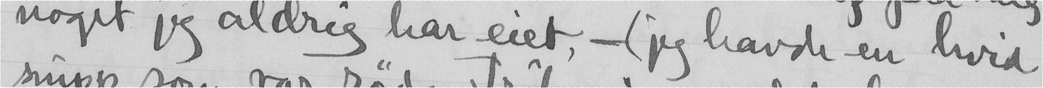noget jeg aldrig har eiet, - (jeg havde en hvid
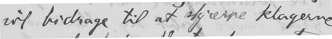vil bidrage til at skjærpe klagerne
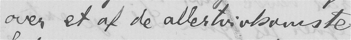over et af de allertvivlsomste
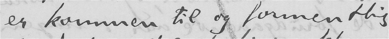er kommen til og formentlig
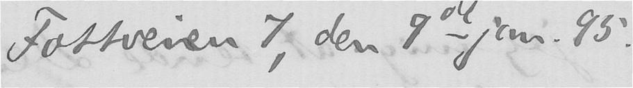Fossveien 7, den 9de jan. 95.
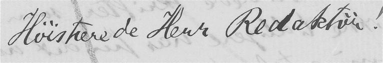Høistærede Herr Redaktør!
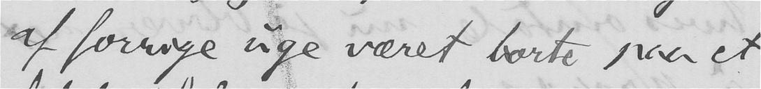af forrige uge været borte paa et
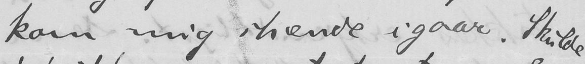kom mig ihænde igaar. Skulde
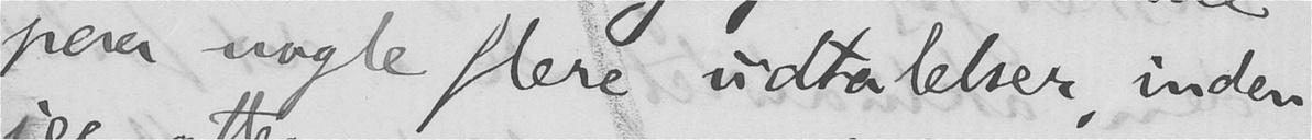paa nogle flere udtalelser, inden
